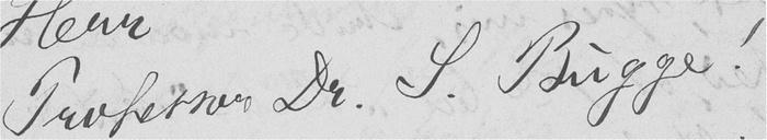Professor Dr. S. Bugge!
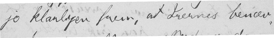jo klarligen frem, at Irernes benæv-
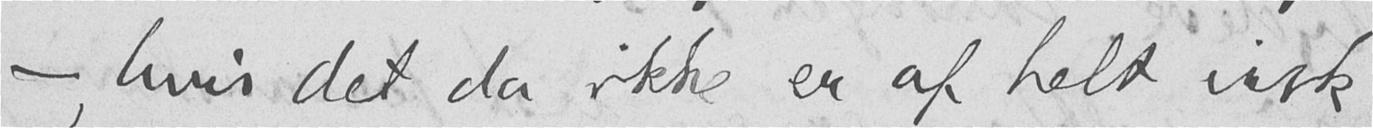- hvis det da ikke er af helt irsk
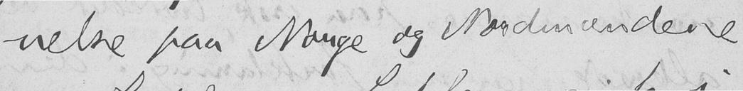nelse paa Norge og Nordmændene
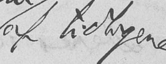af tidligere
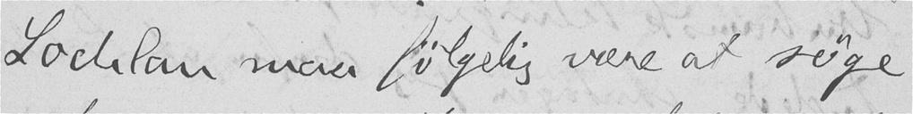Lochlan maa følgelig være at søge
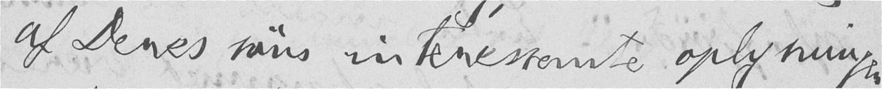af Deres søns interessante oplysninger
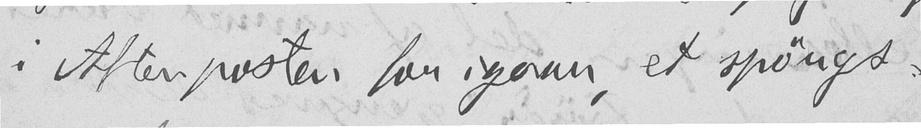i Aftenposten for igaar, et spørgs-
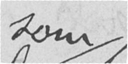som
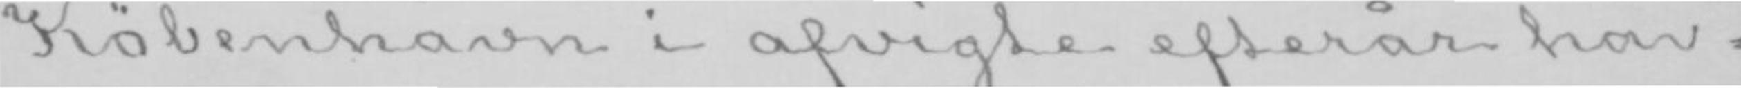København i afvigte efterår hav-
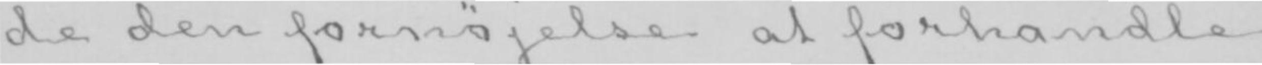de den fornøjelse at forhandle
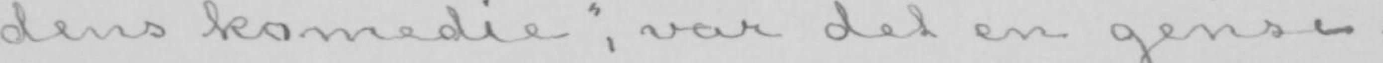dens komedie», var det en gensi-
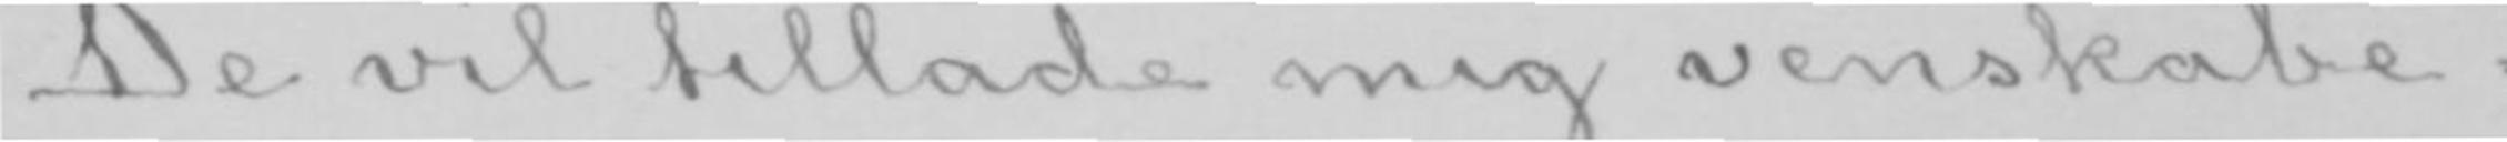De vil tillade mig venskabe-
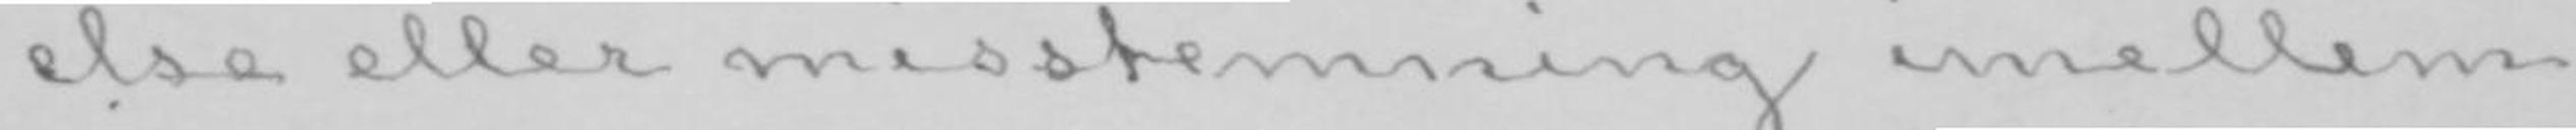else eller misstemning imellem
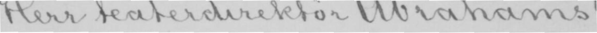Herr teaterdirektør Abrahams!
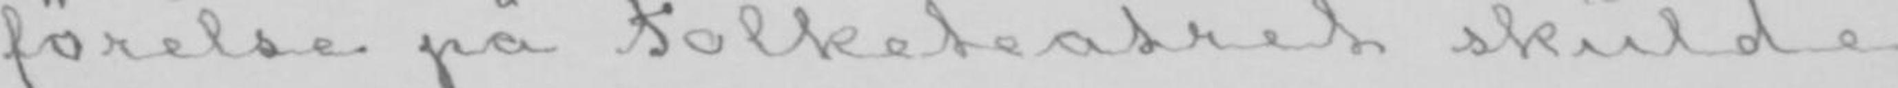førelse på Folketeatret skulde
